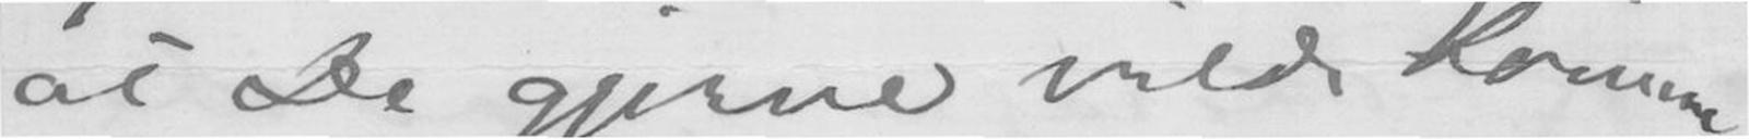at De gjerne vilde Komme
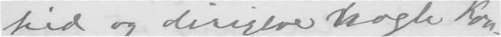hid og dirigere nogle Kon-
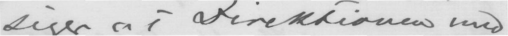siger at Direktionen med
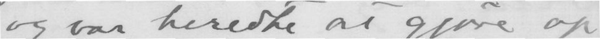og var beredte at gjøre op
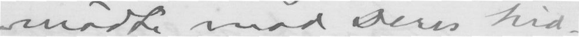mødte med Deres hid-
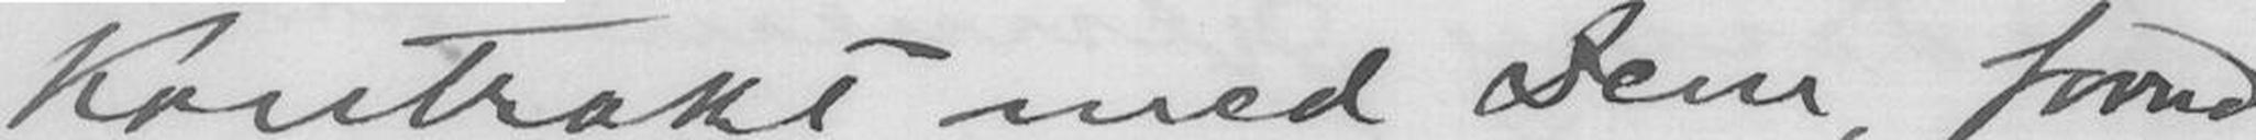Kontrakt med Dem, forud-
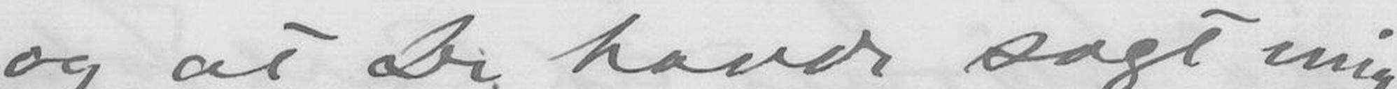og at De havde sagt mig,
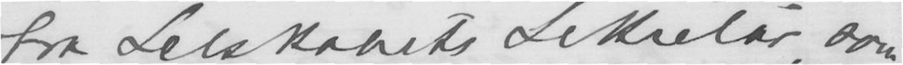fra Selskabets Sekretær, som
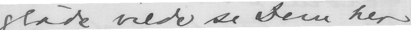glæde vilde se Dem her
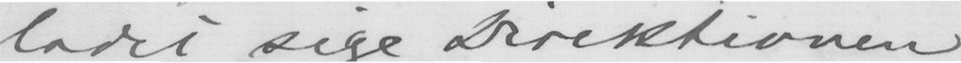ladt sige Direktionen
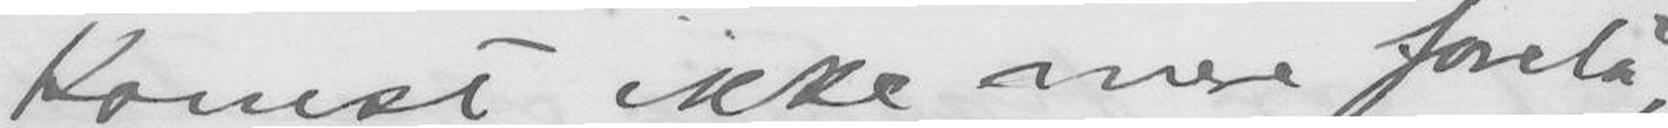komst ikke mere forelå,
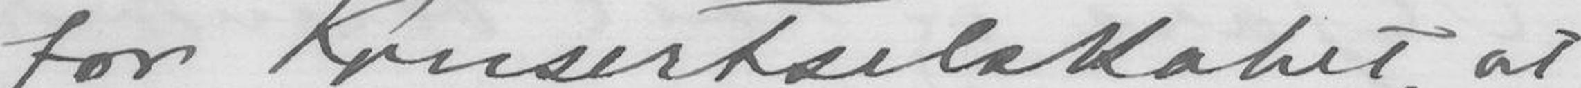for Konsertselskabet, at
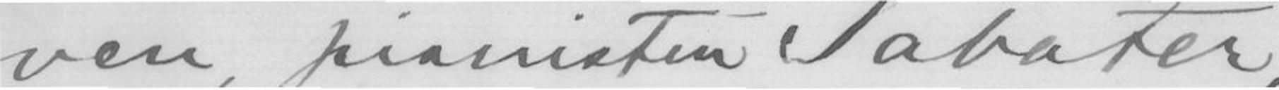ven, pianisten Sabater,
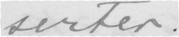serter.
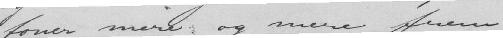toner mere og mere frem
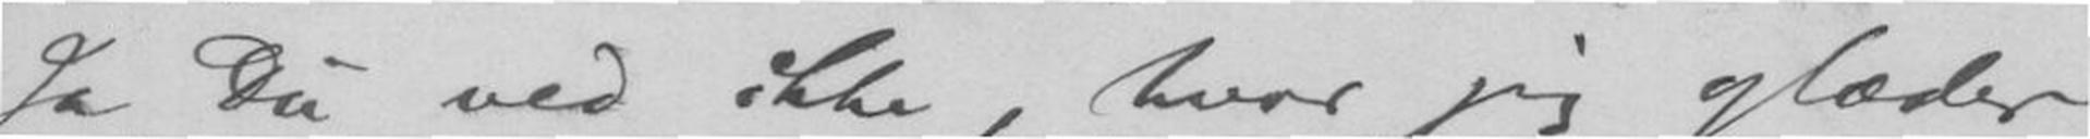Ja Du ved ikke, hvor jeg glæder
